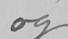og
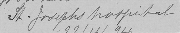St. Josephs hospital
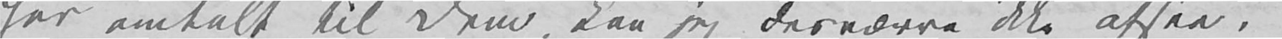har omtalt til Dem, kan jeg desværre ikke afsee.
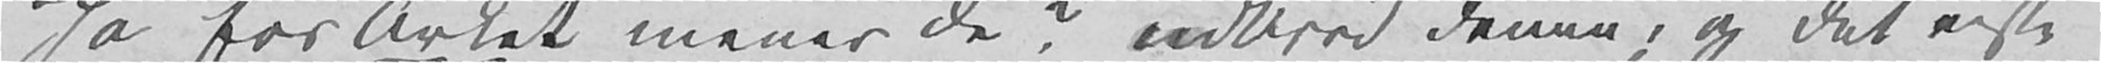«Ja for Arket mener De»? udbrød denne; og det viste
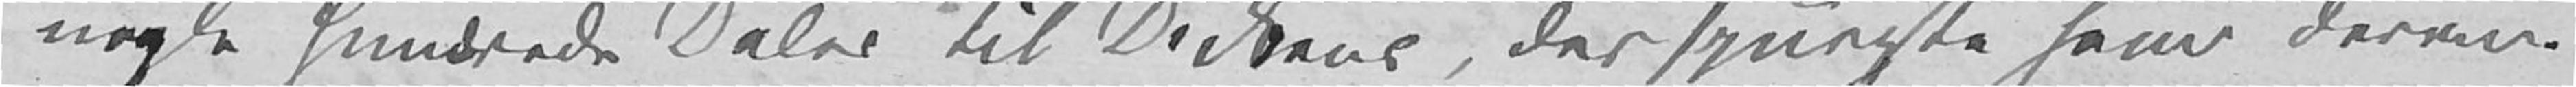nogle hundrede Daler til Dickens, der spurgte ham derom.
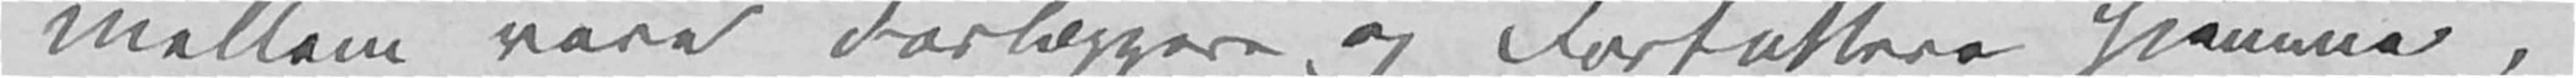mellem vore Forlæggere og Forfattere hjemme,
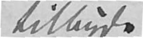tilbyde
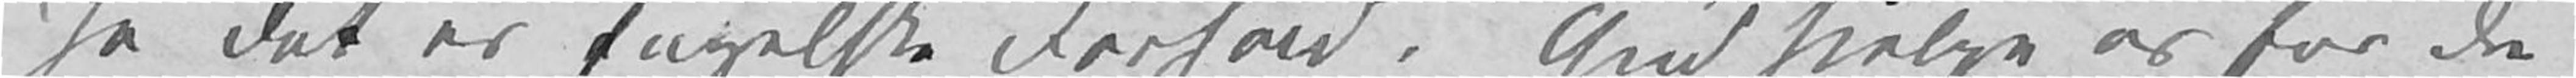Ja det er Engelske Forhold; Gud hjelpe os for de
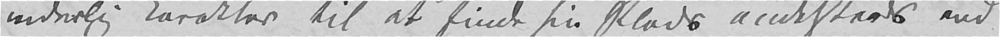inderlig Karakter til at finde sin Plads andetsteds end
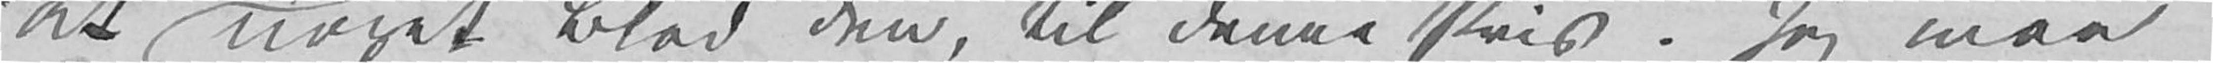at noget Blad den, til denne Pris. Jeg maa
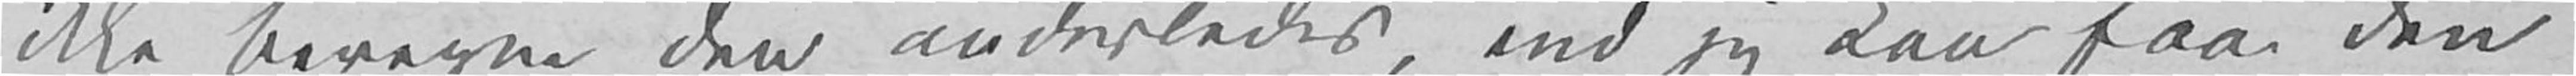ikke beregne den anderledes, end jeg kan faa den
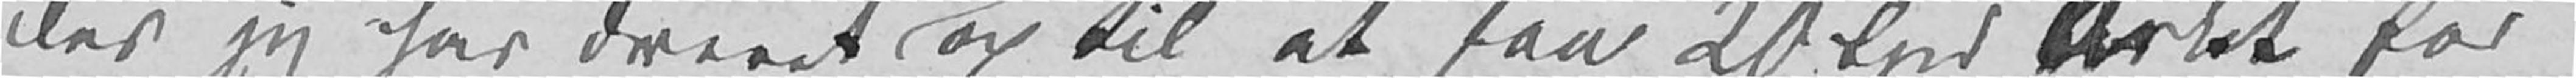der jeg har drevet op til at faa 20 Spd Arket for
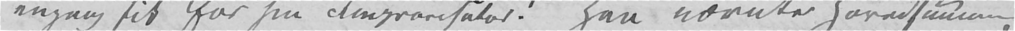engang fik for sin Improvisator. Han nævnte Hovedsummen,
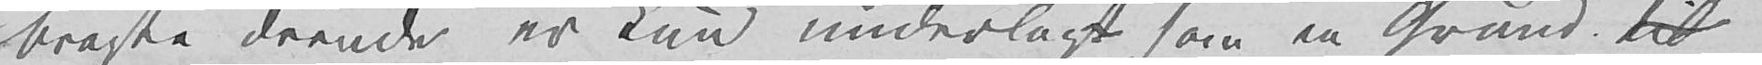bragte derude er kun underlagt som en Grund til
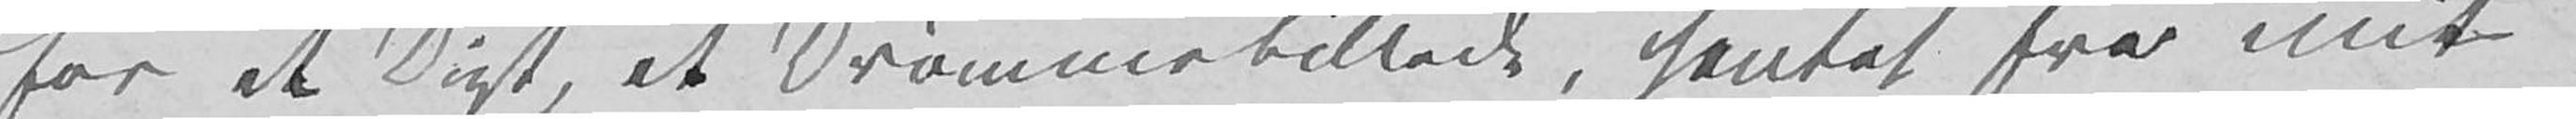for et Digt, et Drømmebillede, hentet fra mit
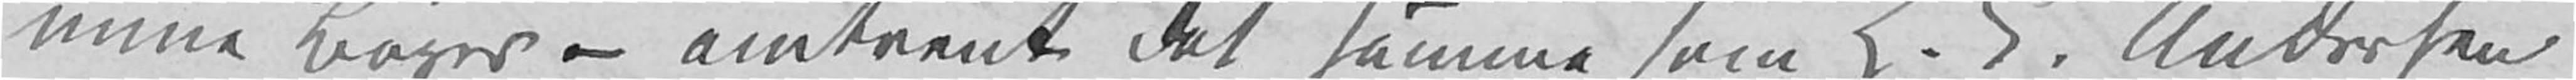mine Bøger – omtrent det samme som H. C. Andersen
